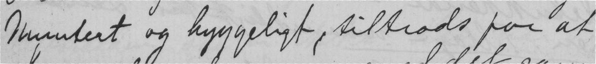Muntert og hyggeligt, tiltrods for at
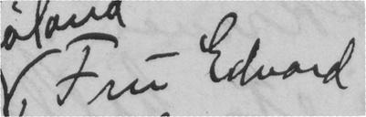, Fru Edvard
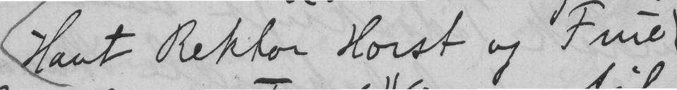Havt Rektor Horst og Frue
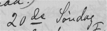20de Søndag
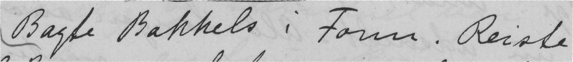Bagte Bakkels i Form. Reiste
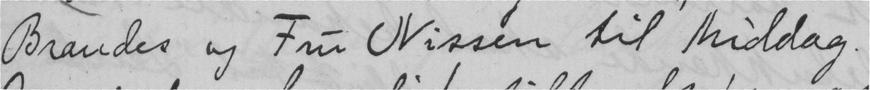Brandes og Fru Nissen til Middag.
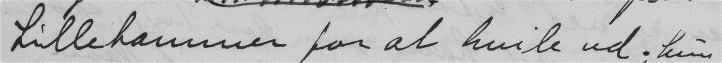Lillehammer for at hvile ud; hun
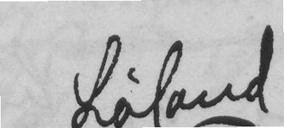Løland
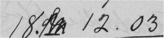18. 12.03
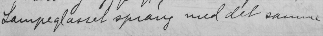Lampeglasser sprang med det samme
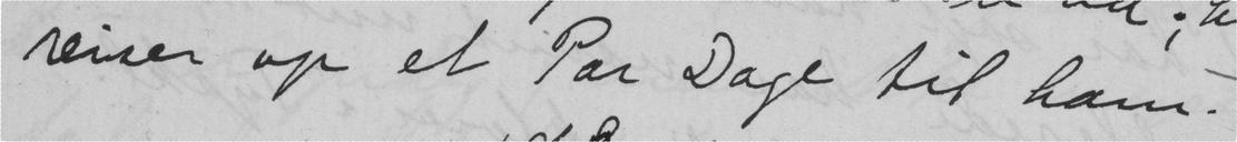reiser op et Par Dage til ham.
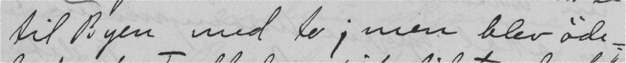til Byen med to; men blev øde-
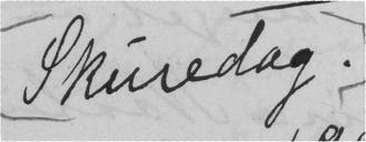Skuredag.
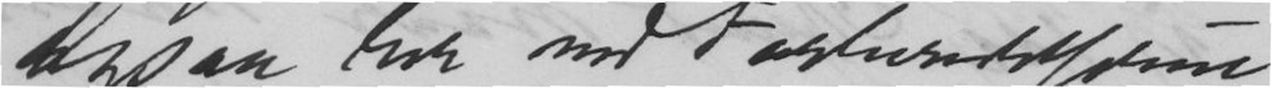ogsaa har ved Forberedelserne
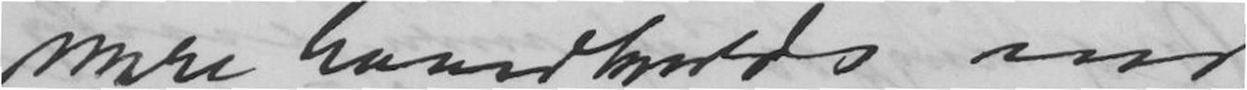mere hovedkulds end
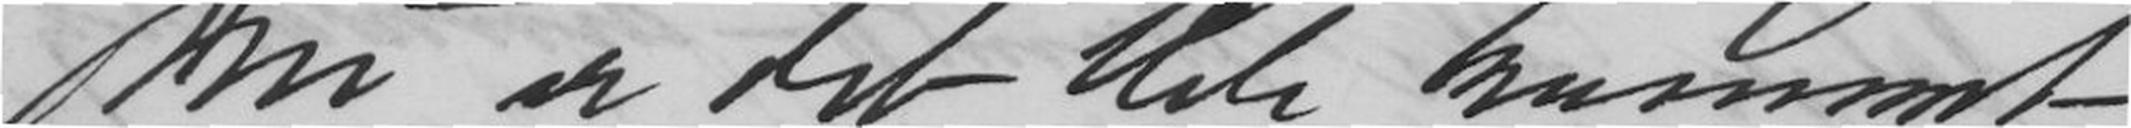Nu er det Hele kommet
